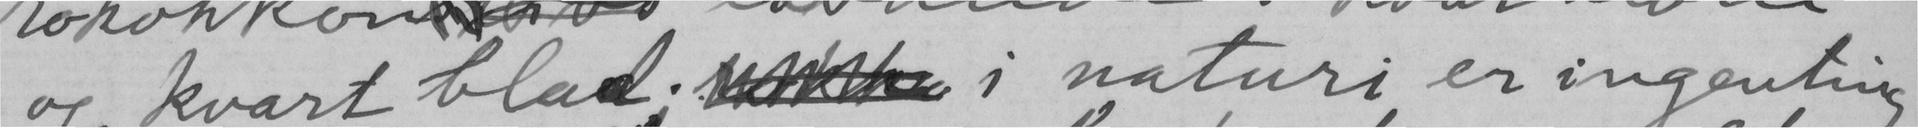og kvart blad. i naturi er ingenting
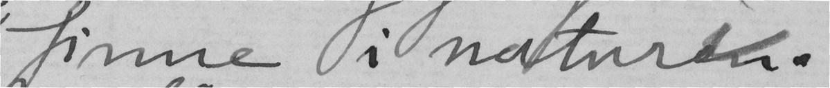finne i naturen.
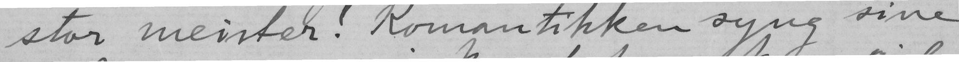stor meister! Romantikken syng sine
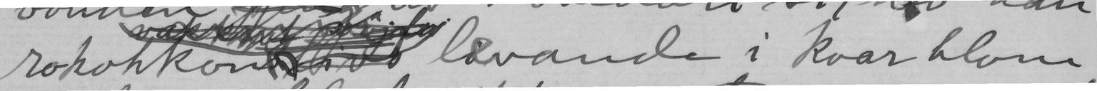rokokkon livande i kvar blom
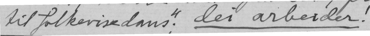til folkevisedans." dei arbeider!
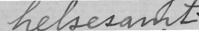helsesamt
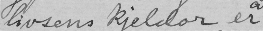livsens kjeldor er
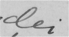dei
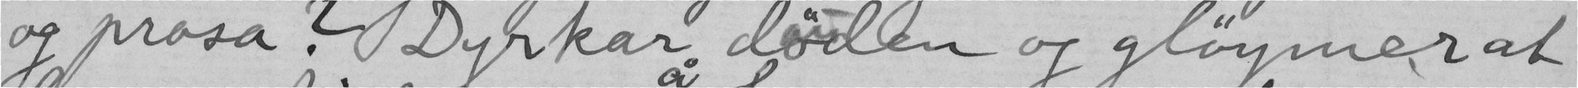og prosa? Dyrkar døden og gløymer at
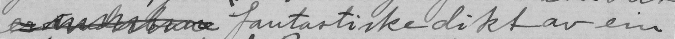fantastiske dikt av ein
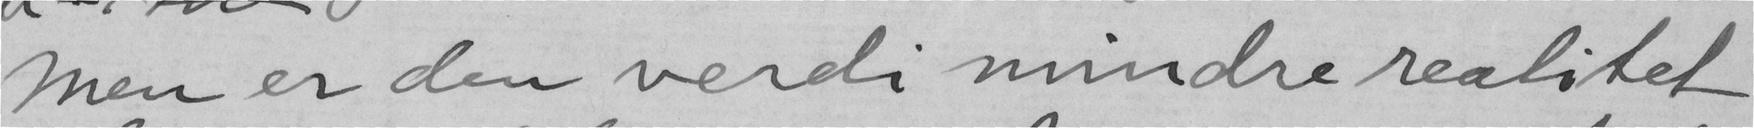Men er den verdi mindre realitet
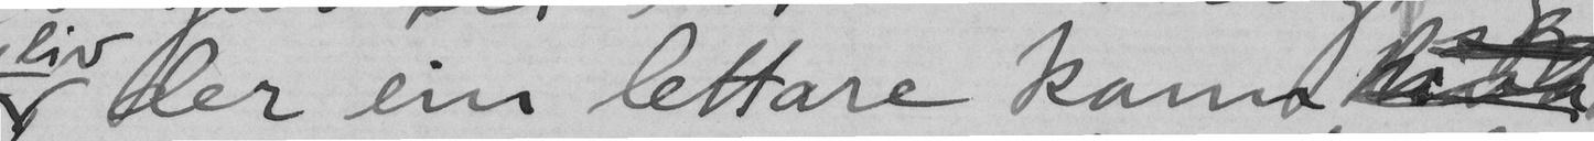der ein lettare kann
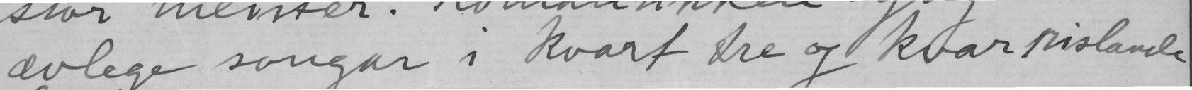ævlege songar i kvart tre og kvar rislande
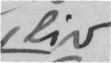liv
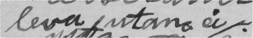leva utan å
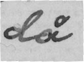då
